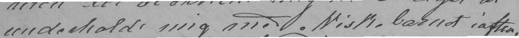underholde mig med Niskebarnet iaften,
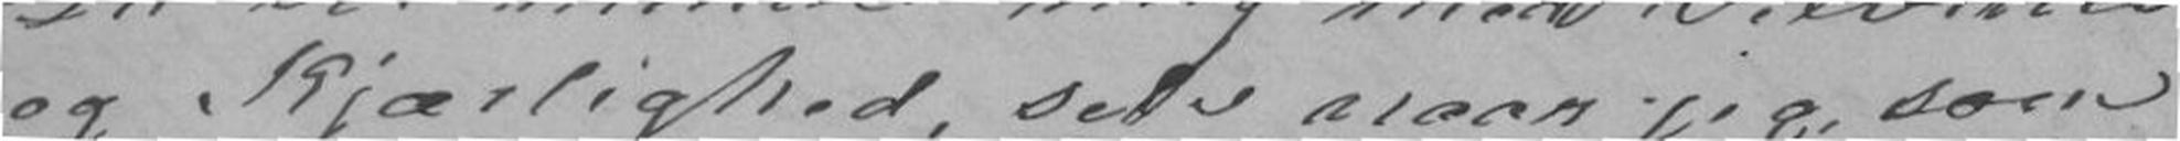og Kjærlighed, selv naar jeg, som
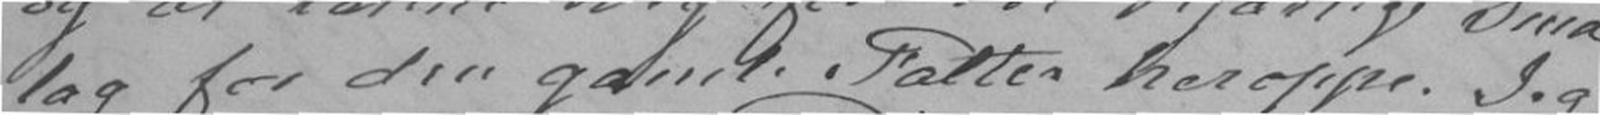lag for din gamle Fatter heroppe. Jeg
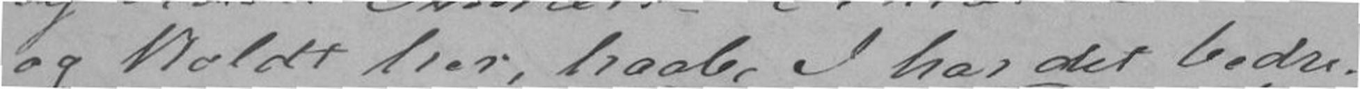og koldt her, haabe I har det bedre.
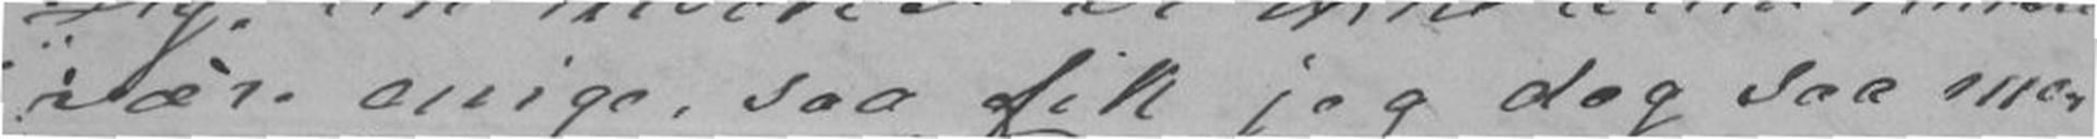være enige, saa fik jeg deg saa me-
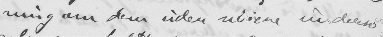ning om dem uden nøiere undersø-
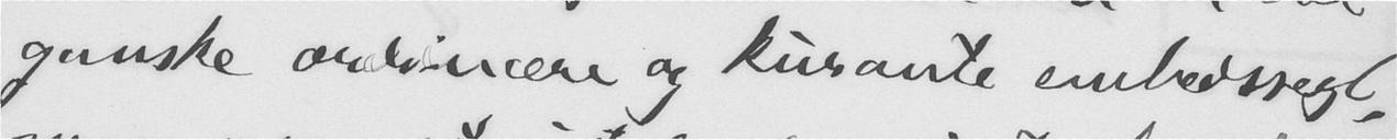ganske ordinære og kurante embedssegl,
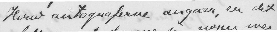Hvad autograferne angaar, er det
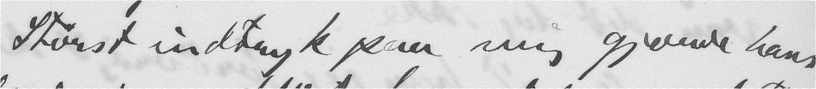Størst indtryk paa mig gjorde hans
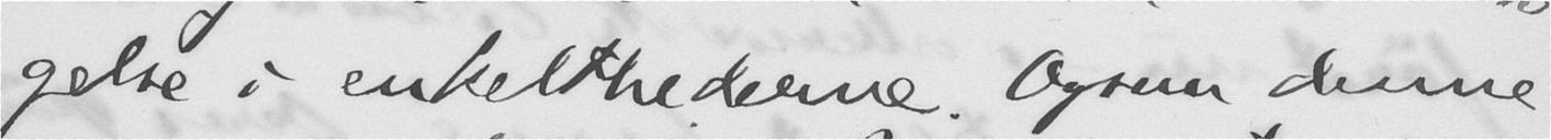gelse i enkelthederne. Ogsaa denne
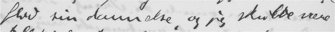flid sin dannelse, og jeg skulde være
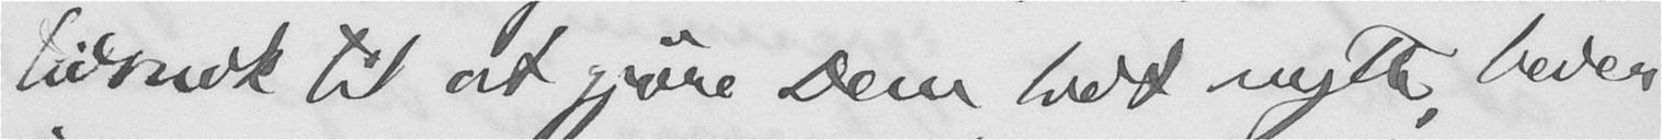tidsnok til at gjøre Dem lidt nytte, beder
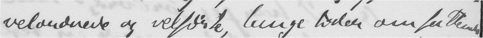velordnede og velførte, lange tider omfattende
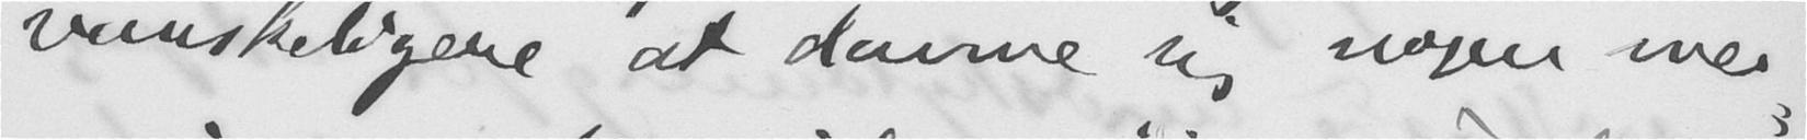vanskeligere at danne sig nogen me-
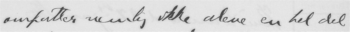omfatter nemlig ikke alene en hel del
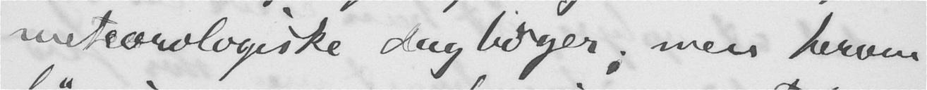meteorologiske dagbøger; men herom
